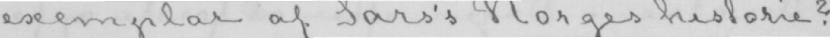exemplar af Sars's Norges historie?
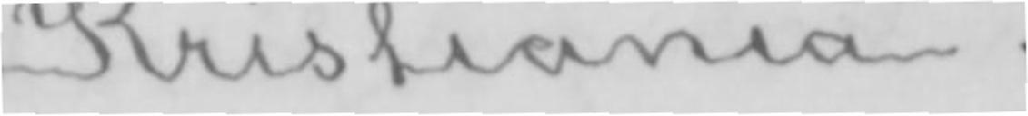Kristiania.
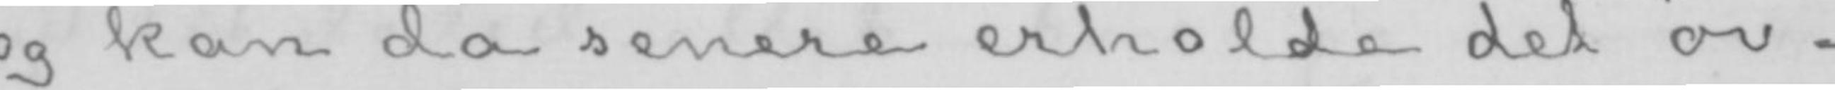og kan da senere erholde det øv-
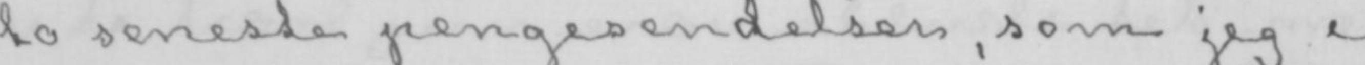to seneste pengesendelser, som jeg i
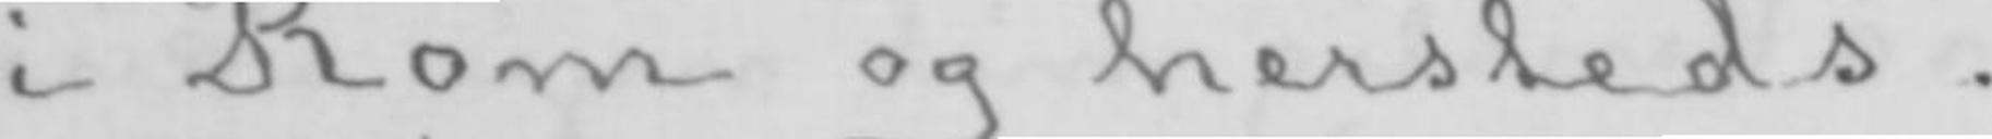i Rom og hersteds.
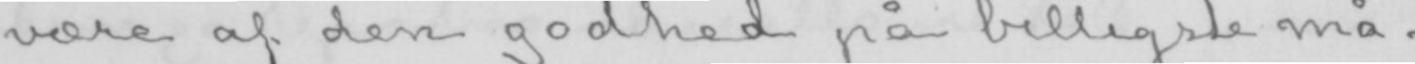være af den godhed på billigste må-
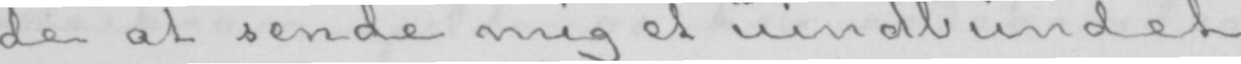de at sende mig et uindbundet
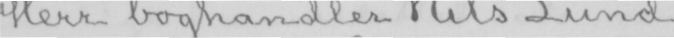Herr boghandler Nils Lund.
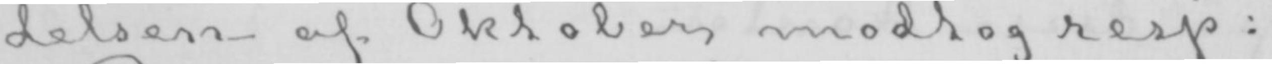delsen af Oktober modtog resp:
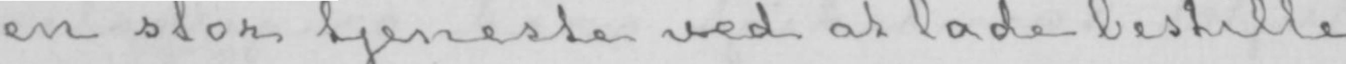en stor tjeneste ved at lade bestille
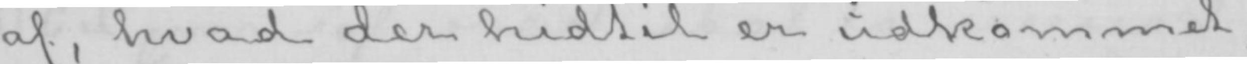af, hvad der hidtil er udkommet,
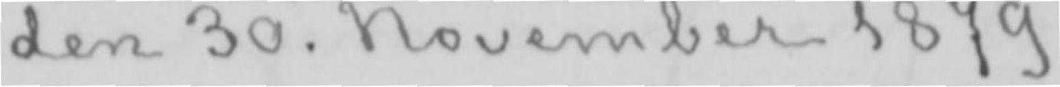den 30. November 1879.
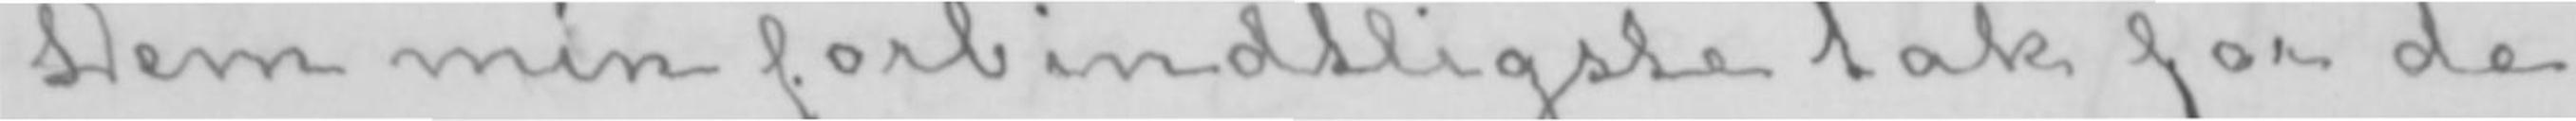Dem min forbindtligste tak for de
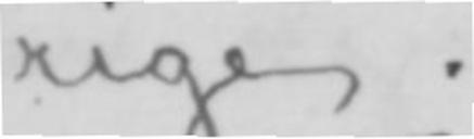rige.
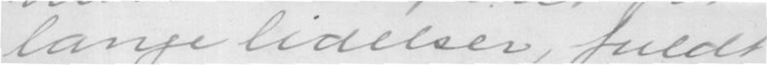lange lidelser, fuldt
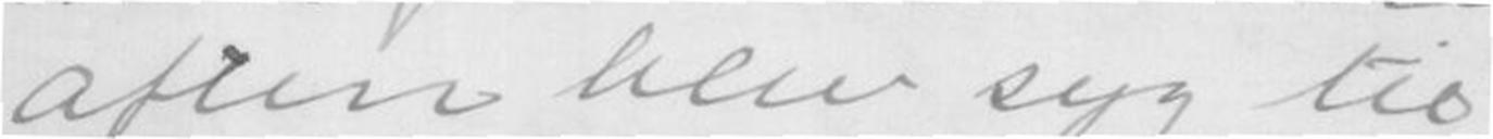aften blev syg til
